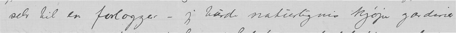selv til en forlægger – jeg burde naturligvis kjøpe gardiner
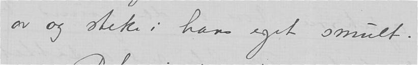av og steke i hans eget smult.
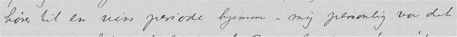hører til en viss periode hjemme – mig personlig var det
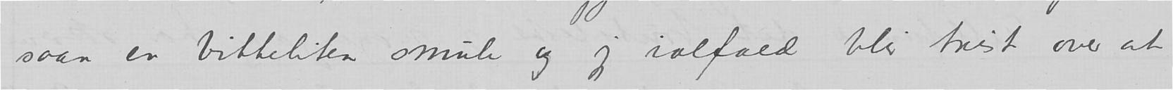saan en bitteliten smule og jeg ialfald blir trist over at
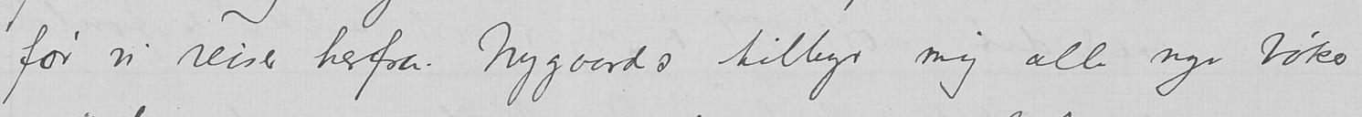før vi reiser herfra. Nygaards tilbyr mig alle nye bøker
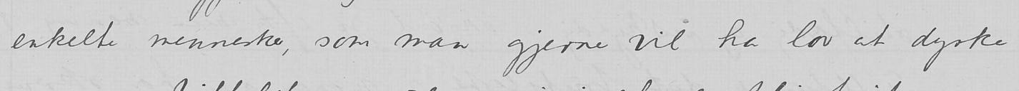enkelte mennesker, som man gjerne vil ha lov at dyrke
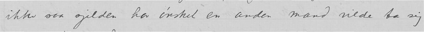ikke saa sjelden har ønsket en anden mand vilde ta sig
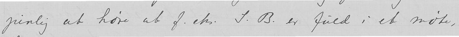pinlig at høre at f.eks. S.B. er fuld i et møte,
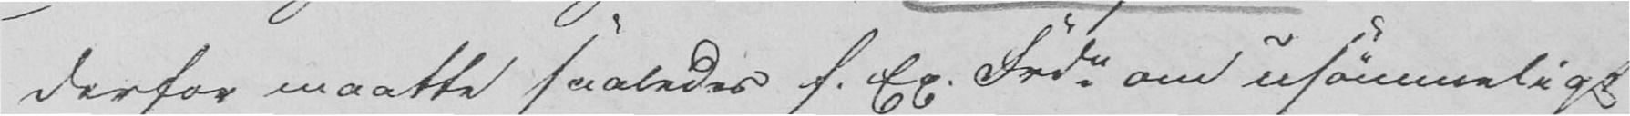derfor maatte saaledes f. Ex. Frdn om usømmeligt
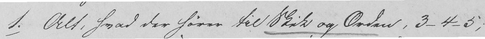1. Alt, hvad der hører til Skik og Orden, 3-4-5;
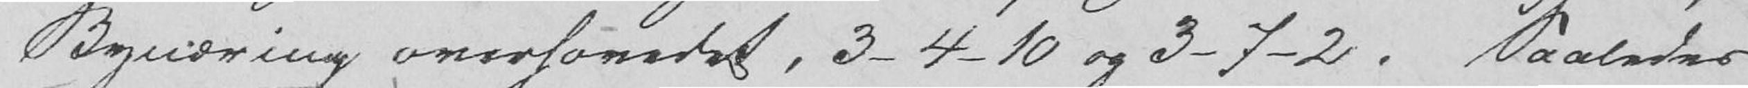Bynæring overhovedet, 3-4-10 og 3-7-2. Saaledes
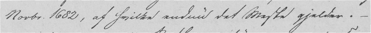Novbr. 1682, af hvilke endnu det Meste gjelder. -
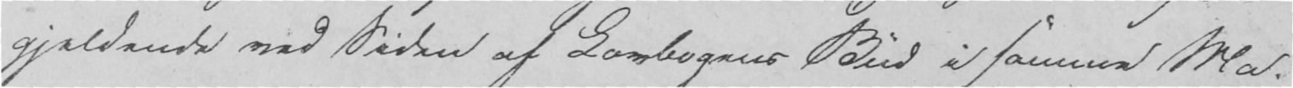gjeldende ved Siden af Lovbogens Bud i samme Ma-
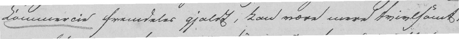Commercie fremdeles gjaldt, kan være mere tvivlsomt,
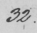32.
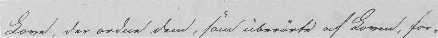Love, der ordne dem, som uberørte af Loven, for-
